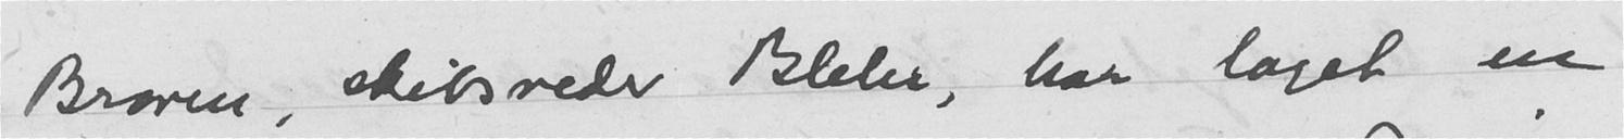Broren, skibsreder Blehr, har laget en
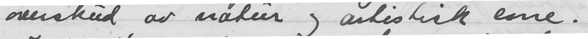overskud av natur og artistisk lone.
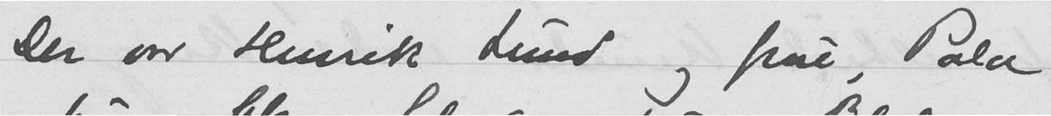Der er Henrik Lund og frue, Pola
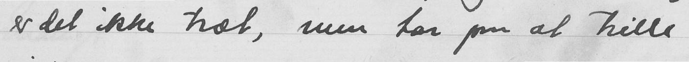er det ikke træt, men tar paa at trille
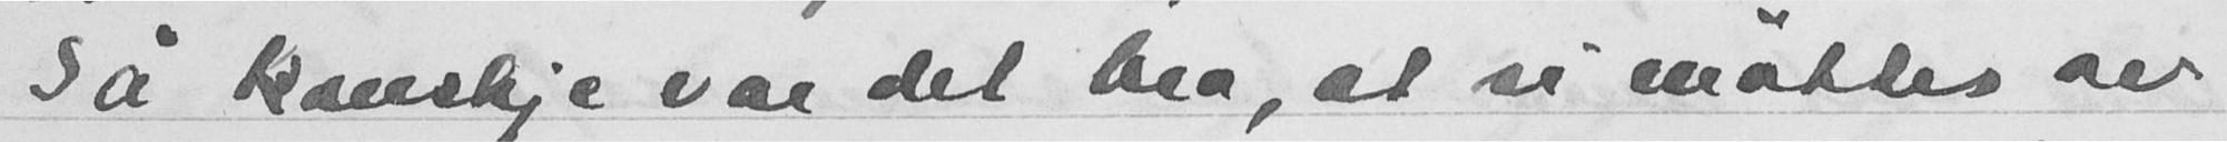Så kanskje var det bra, at vi møttes der
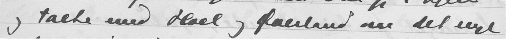og talte med Hoel og Øverland om det nye
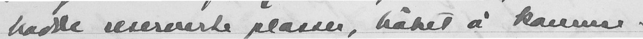hadde reservert plasser, håpet å komme.
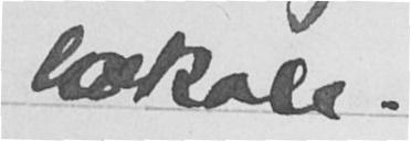lokale.
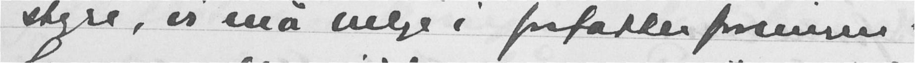styre, vi må velge i forfatterforeningen.
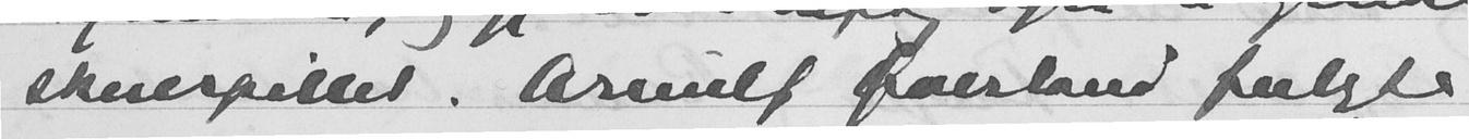skuespillet. Arnulf Øverland fulgte
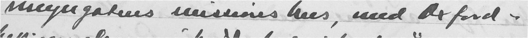meyergatens missions hus, med Oxford-
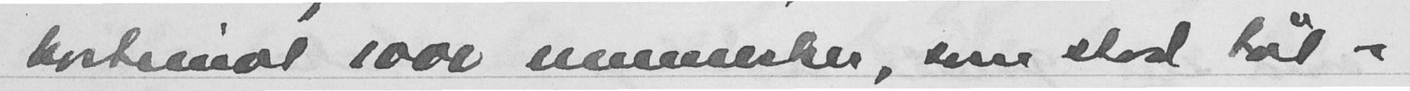Bortimot 100e mennesker, som stod tål-
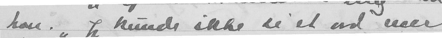han. "Jeg kunde ikke si et ord mer
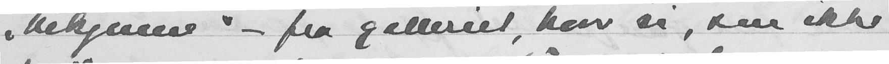"bekjenne" - fra galleriet, kom vi, som ikke
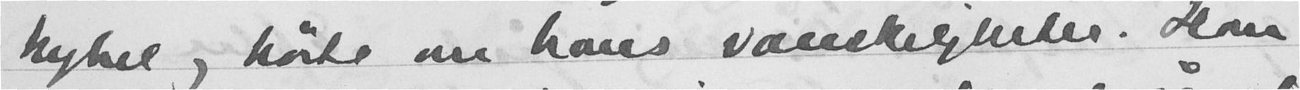hybel og hørte om hans vanskeligheter. Han
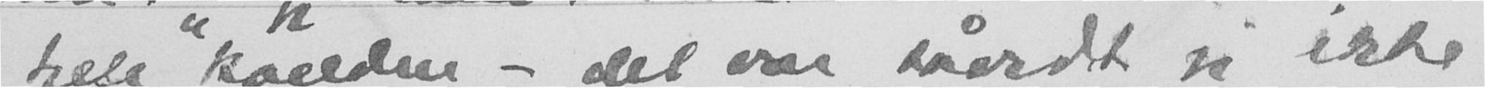hele kvelden - det var såvidt jeg ikke
